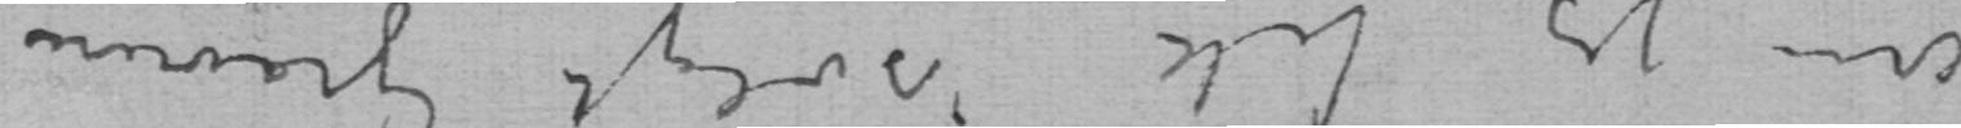om jeg fik solgt Gravurene
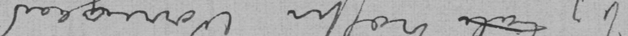Jeg tale træffer Nørregaard
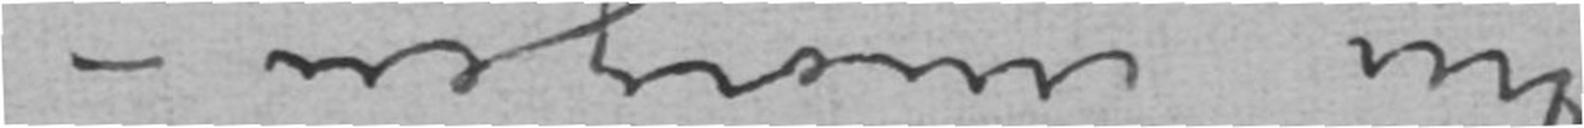her imorgen –
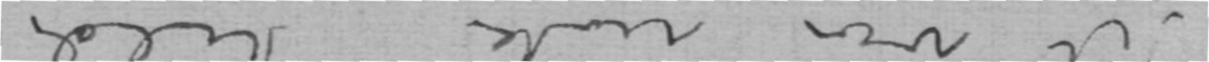Det var nok heldi
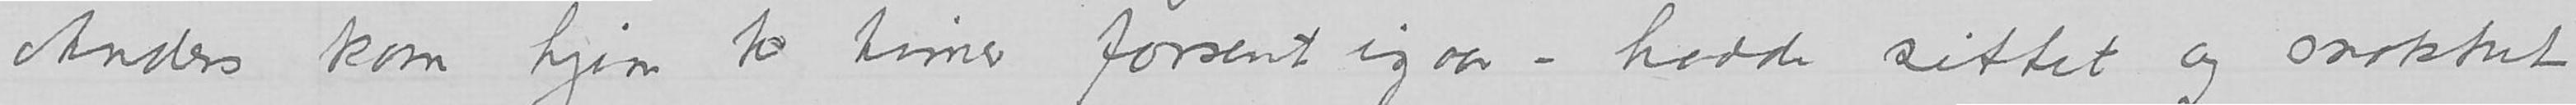Anders kom hjem to timer forsent igaar – hadde sittet og snakket
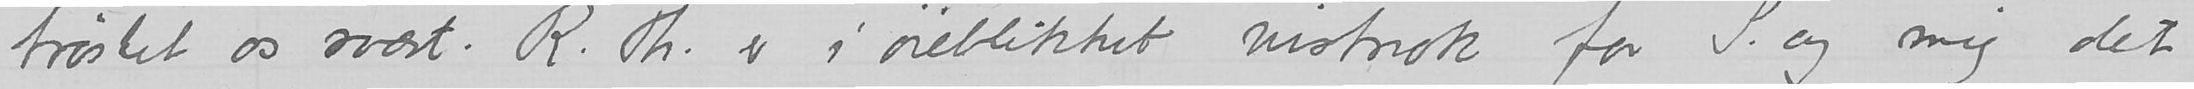trøstet os svært. R.Th. er i øieblikket vistnok for S. og mig det
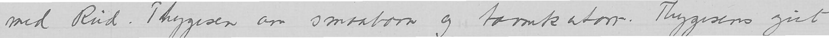med Rud. Thygesen om smaabarn og tarmkatarr. Thygesens gut
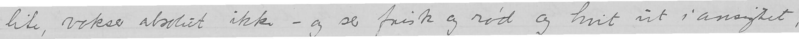lite, vokser absolut ikke – og ser frisk og rød og hvit  ut i ansigtet,
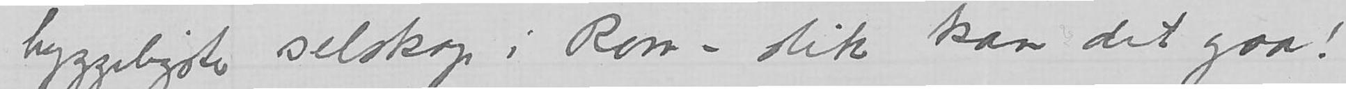hyggeligste selskap i Rom – slik kan det gaa!
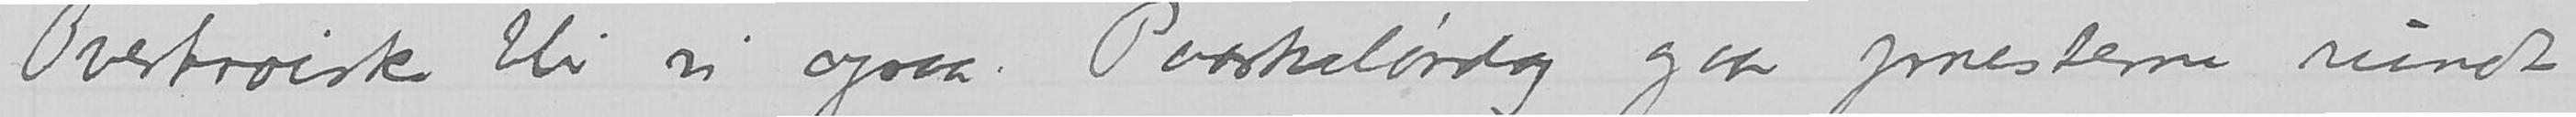Overtroisk blir vi ogsaa. Paaskelørdag gaar presterne rundt
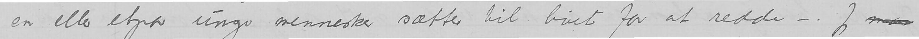en eller etpar unge mennesker sætter til livet for at redde –. Jeg maa
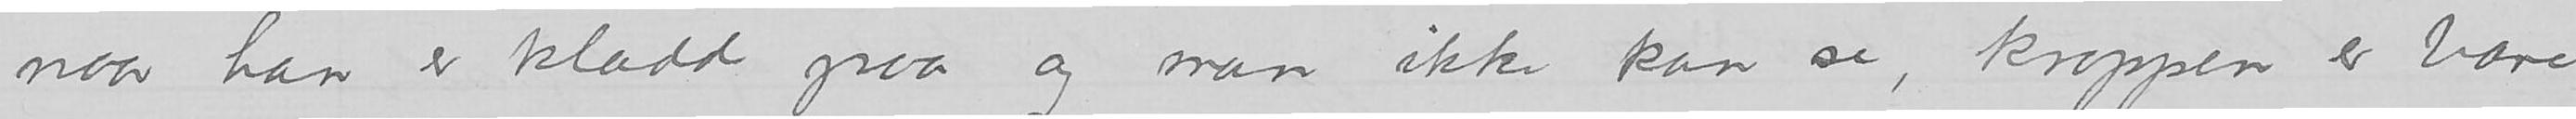naar han er klædd paa og man ikke kan se, kroppen er bare
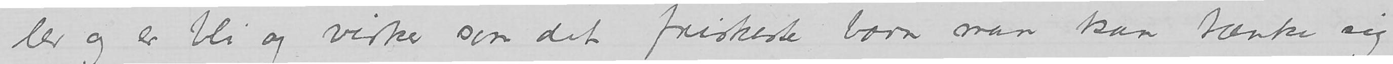ler og er bli og virker som det friskeste barn man kan tænke sig
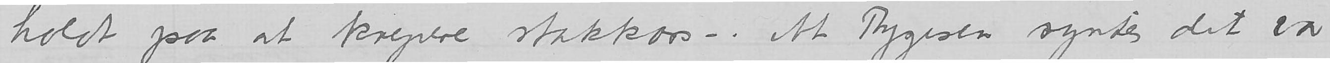holdt paa at krepere stakkars –. At Thygesen syntes det var
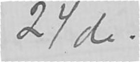24de.
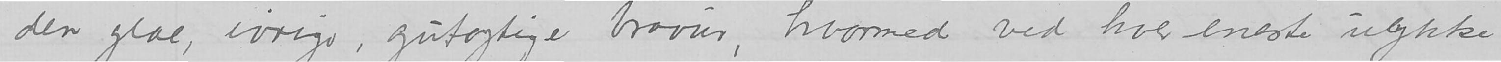den glae, ivrige, gutagtige bravur, hvormed ved hver eneste ulykke
